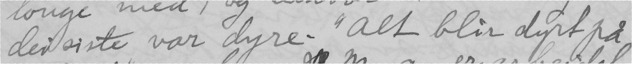dei siste var dyre. "Alt blir dyrt på
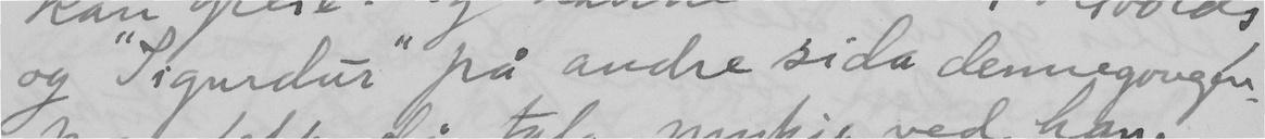og "Sigurdur" på andre sida dennegongen.
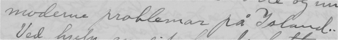moderne problemar på Island.
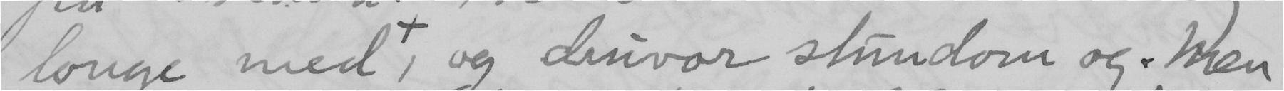longe med, og druvor stundom og, men
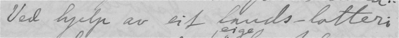Ved hjelp av eit lands-lotteri
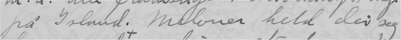på Island. Meloner held dei seg
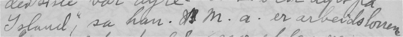Island" sa han. M.a. er arbeidslonene
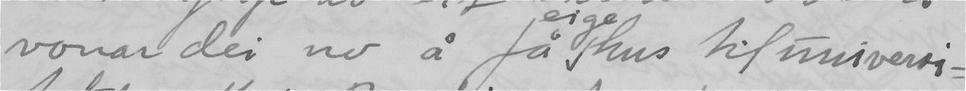vonar dei, no å få eige hus til universi-
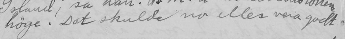höge. Det skulde no elles vera godt.
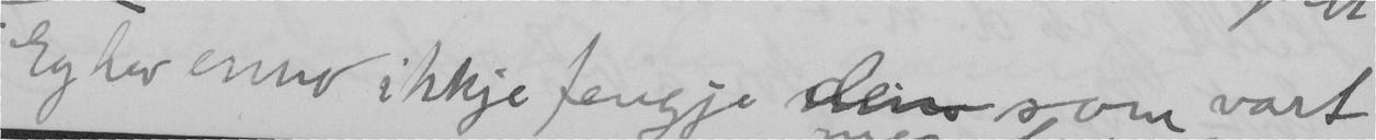Eg hev enno ikkje fengje dei som vart
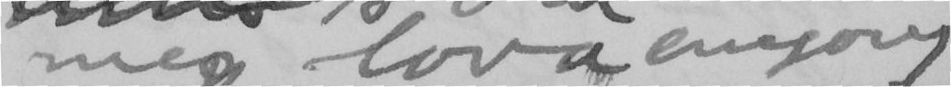meg lova eingong.
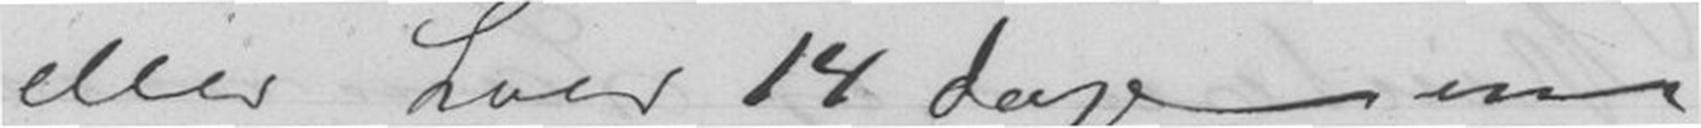eller hver 14 Dage en
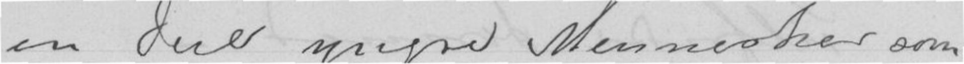en Deel yngre Mennesker som
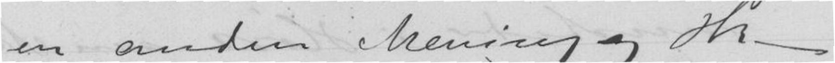en anden Mening og Hr -
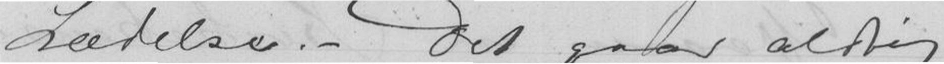Lædelse. - Det gaar aldrig
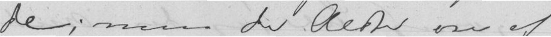de; maa det Altid om af
